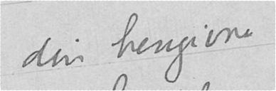din hengivne
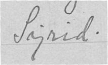Sigrid.
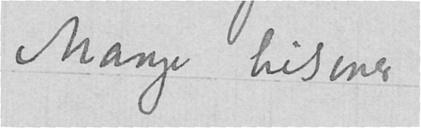Mange hilsener
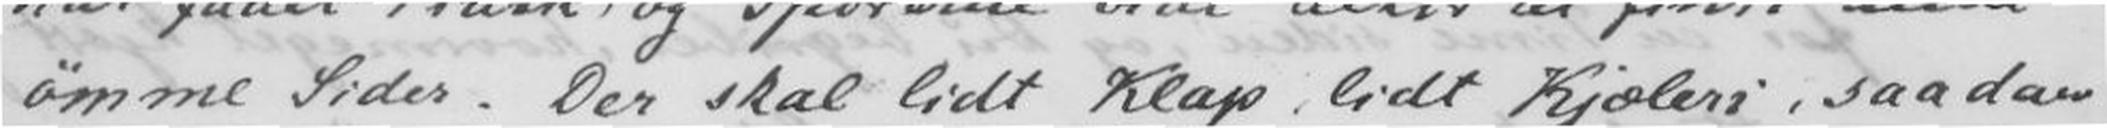ømme Sider. Der skal lidt Klap lidt Kjøleri, saadan
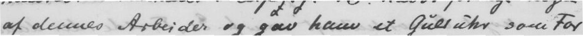af dennes Arbeides og gav ham et Gulduhr som For
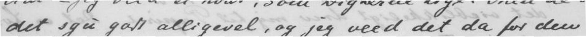det sgu godt alligevel, og jeg veed det da for den
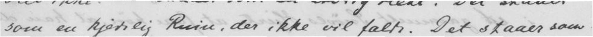som en kjedelig Ruin, der ikke vil falde. Det staaer som
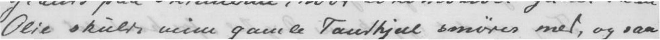Olie skulde mine gamle Tandhjul smøres med, og saa
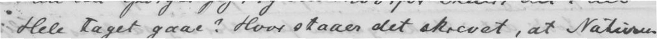Hele taget gaae? Hvor staaer det skrevet, at Naturen
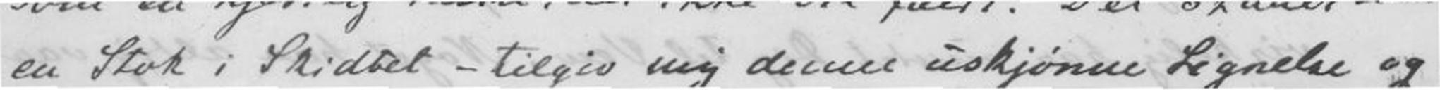en Stok i Skidtet - tilgiv mig denne uskjønne Lignelse og
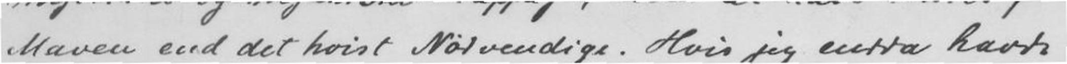Maven end det høist Nødvendige. Hvis jeg endda havde
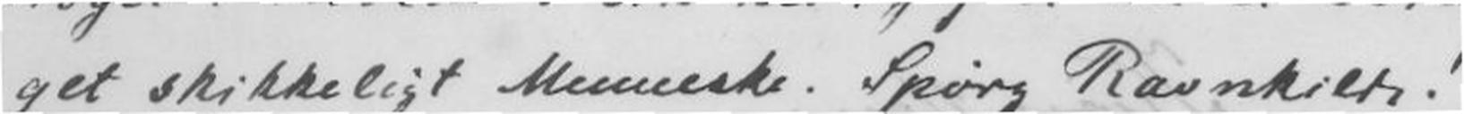get skikkeligt Menneske. Spørg Ravnkilde!
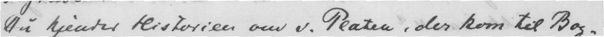Du kjender Historien om v. Plateu, der kom til Bog-
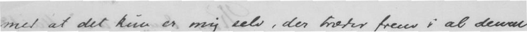med at det kun er mig selv, der træder frem i al denne
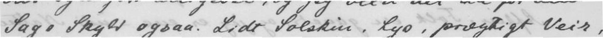Sags Skyld ogsaa. Lidt Solskin, Lys, prægtigt Veir,
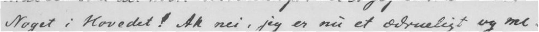Noget i Hovedet! Ak nei, jeg er nu et ordentligt og me-
